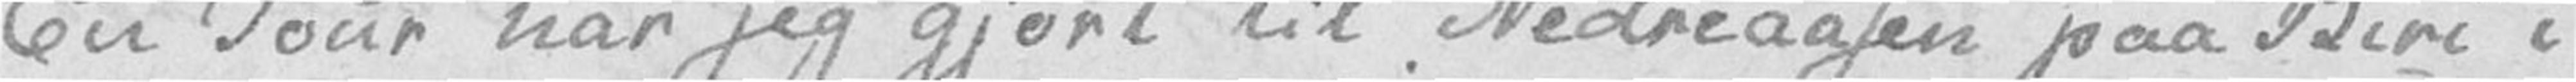En Tour har jeg gjort til Nedreaasen paa Biri i
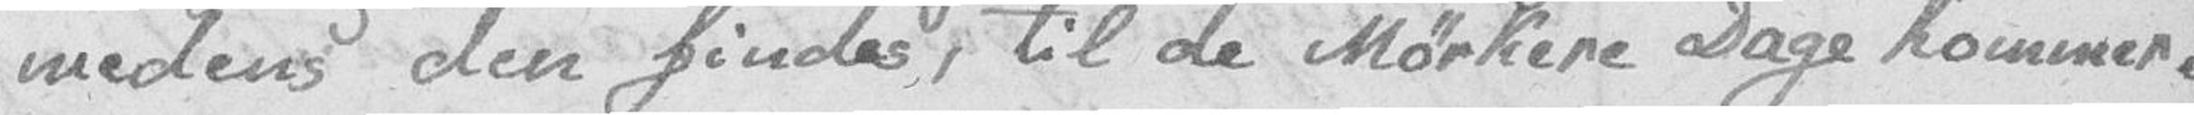medens den findes, til de Mørkere Dage kommer.
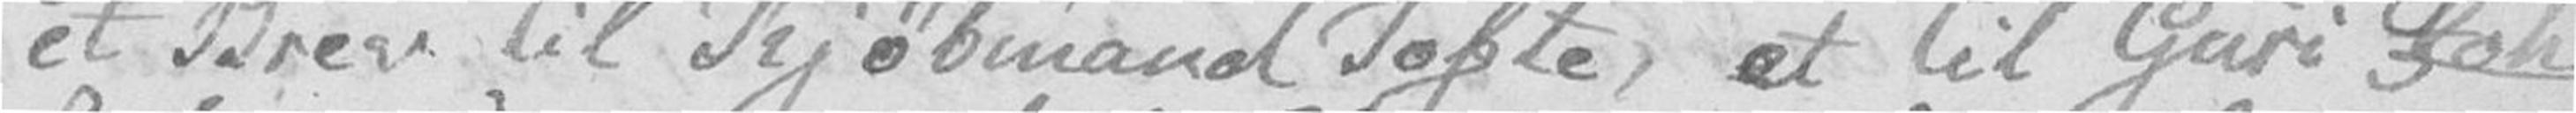et Brev til Kjøbmand Tofte, et til Guri
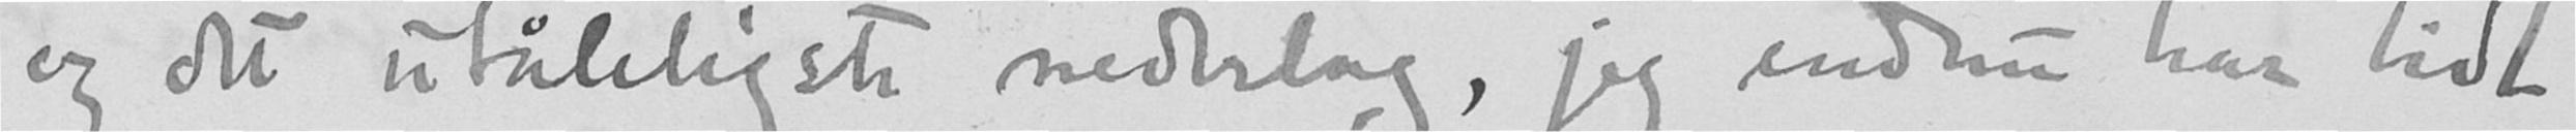og det utåleligste nederlag, jeg endnu har lidt
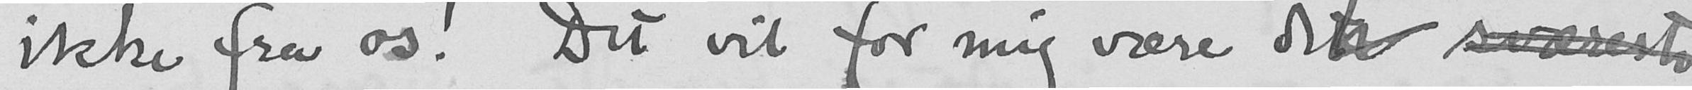ikke fra os! Det vil for mig være det sværeste
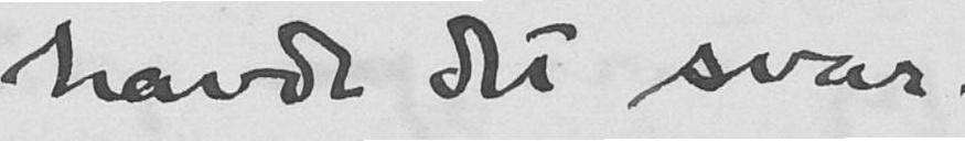havde dit svar.
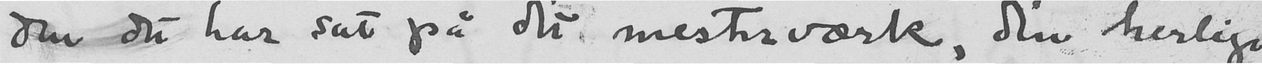den du har sat på dit mesterværk, din herlige
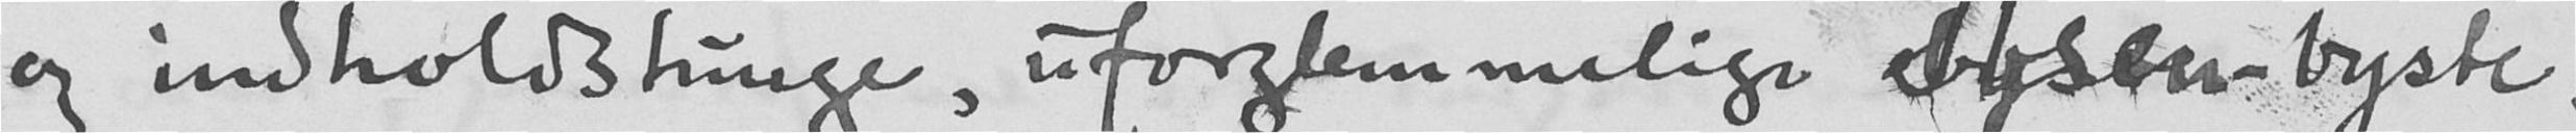og indholdstunge, uforglemmelige Ibsen-byste.
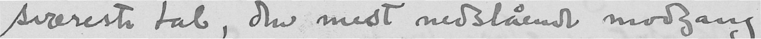sværeste tab, den mest nedslående modgang
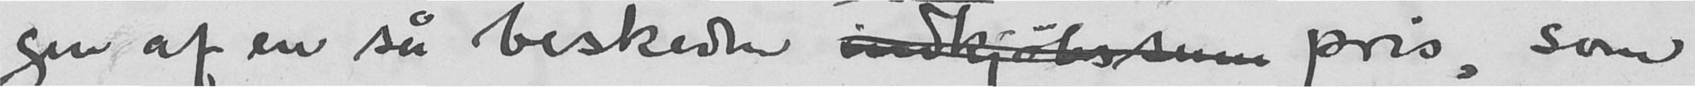gen af en så beskeden indkjøbssum pris, som
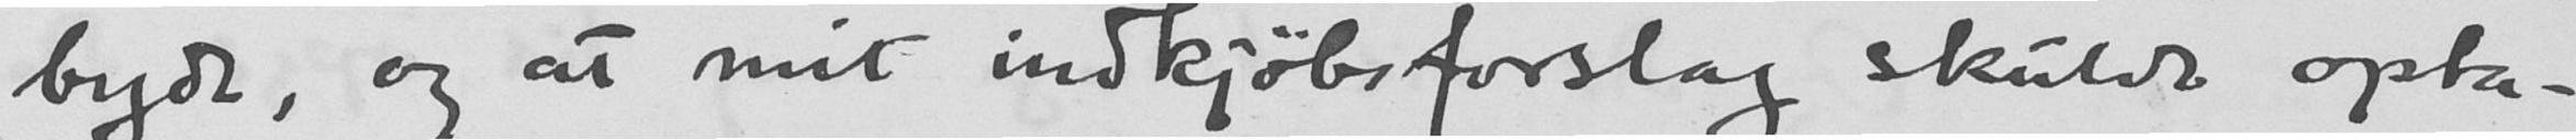byde, og at mit indkjøbsforslag skulde opta-
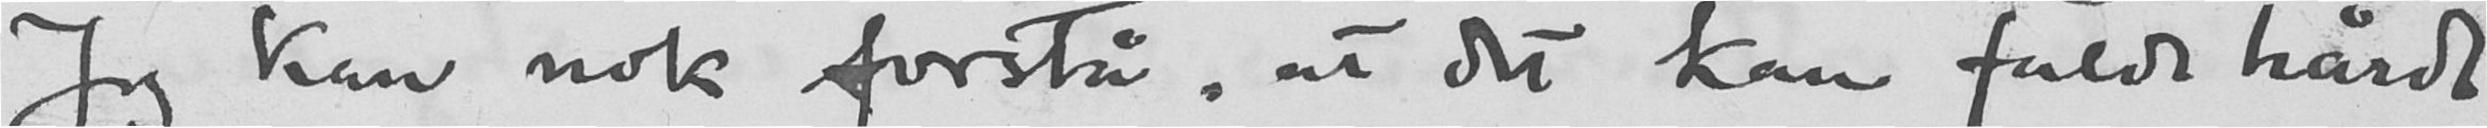Jeg kan nok forstå, at det kan falde hårdt
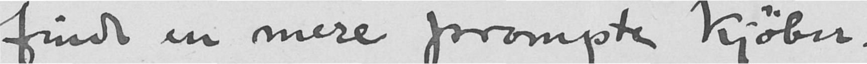finde en mere prompte kjøber.
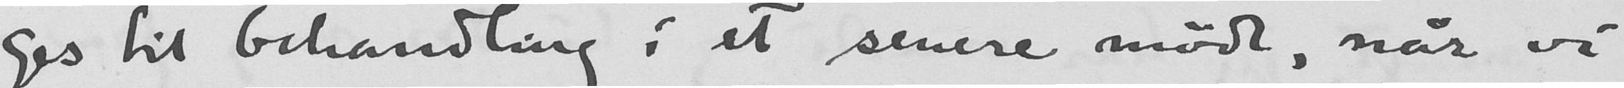ges til behandling i et senere møde, når vi
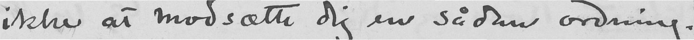ikke at modsætte dig en sådan ordning!
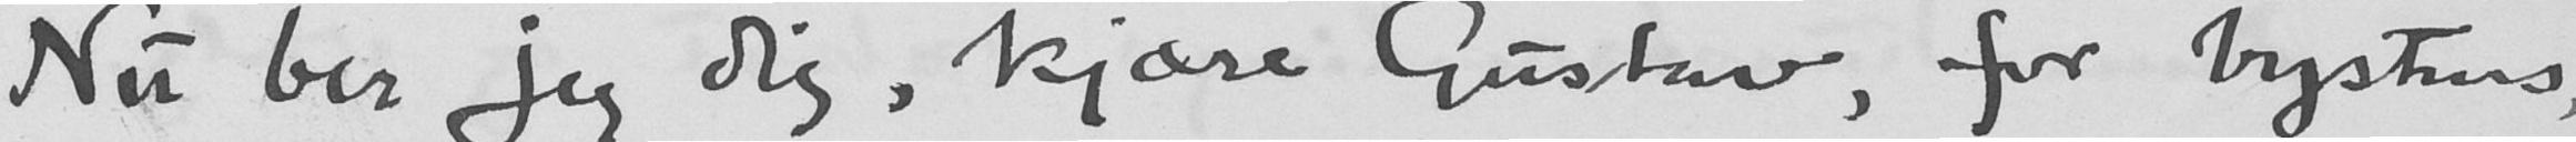Nu ber jeg dig, kjære Gustav for bystens,
